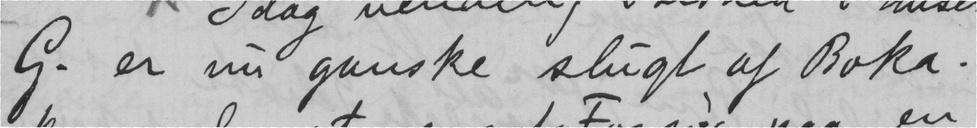G. er nu ganske slugt af Boka.
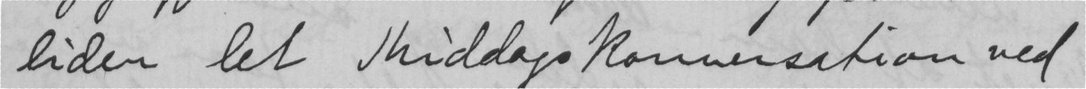liden let Middagskonversation ved
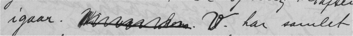i gaar. . V. har samlet
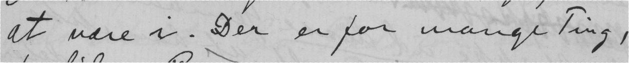at være i. Der er for mange Ting,
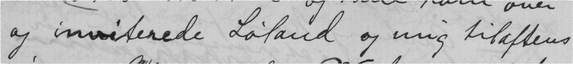og iinviterede Løland og mig til aftens
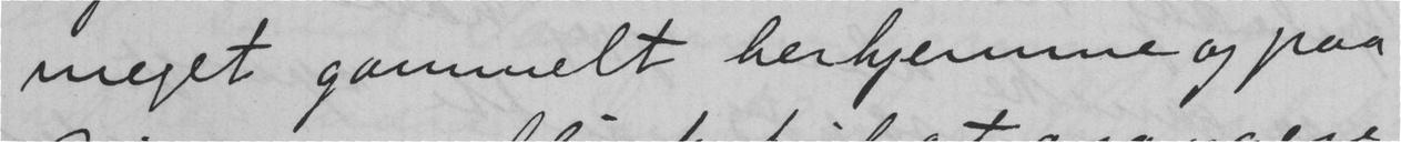meget gammelt herhjemme og paa
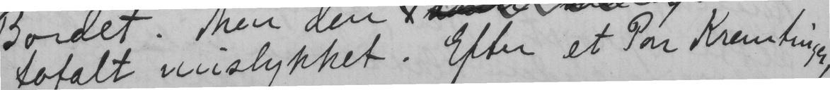totalt mislykket. Efter et Par Kremtinger.
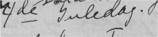4de juledag.
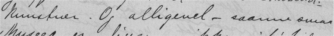kunstner. Og alligevel - saanne smaa
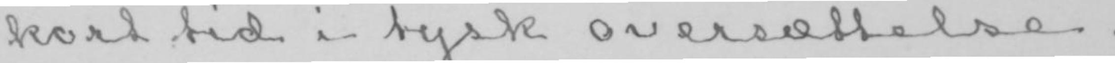kort tid i tysk oversættelse.
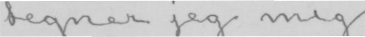tegner jeg mig
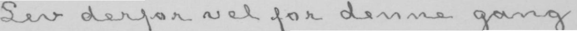Lev derfor vel for denne gang!
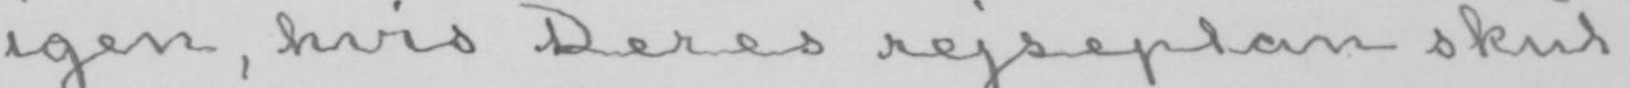igen, hvis Deres rejseplan skul-
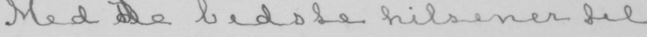Med De bedste hilsener til
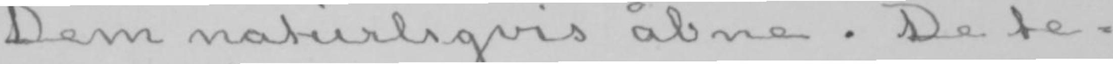Dem naturligvis åbne. De te-
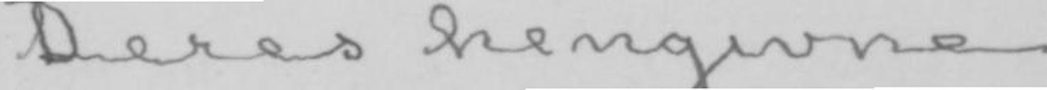Deres hengivne
